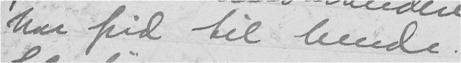har frid til hende.
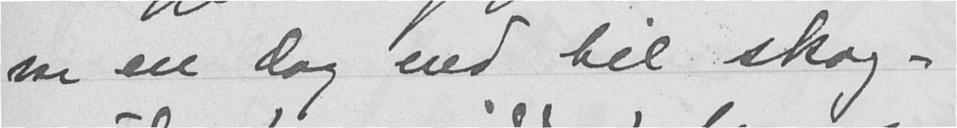var en dag ned til skog-
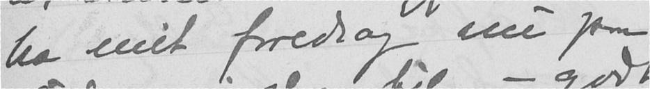ha mit foredrag nu paa
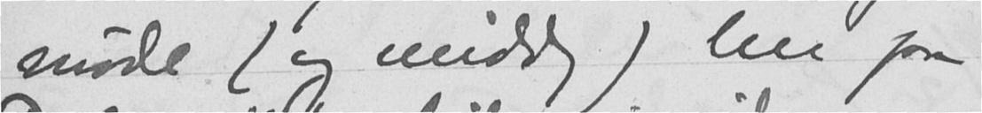møde (og middag) her paa
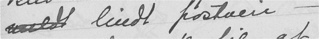meldt lindt frostveir -
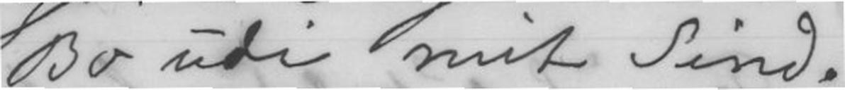Bo udi mit Sind.
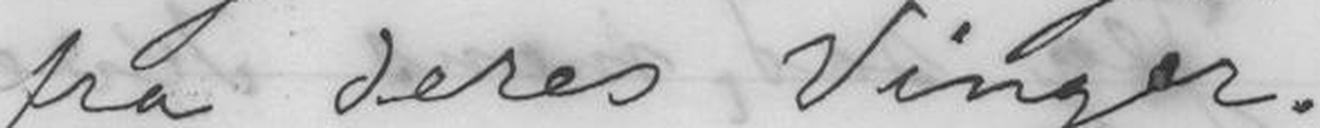fra Deres Vinger.
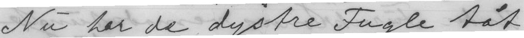Nu har de dystre Fugle tat
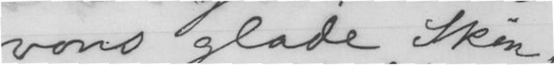vons glade Skin,
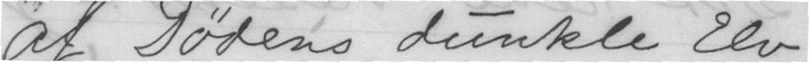af Dødens dunkle Elv
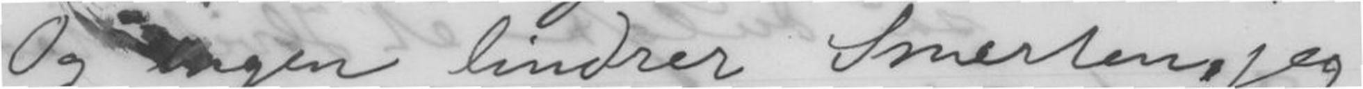Og ingen lindrer Smerten, jeg
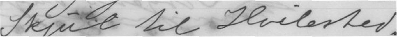Skjul til Hvilested.
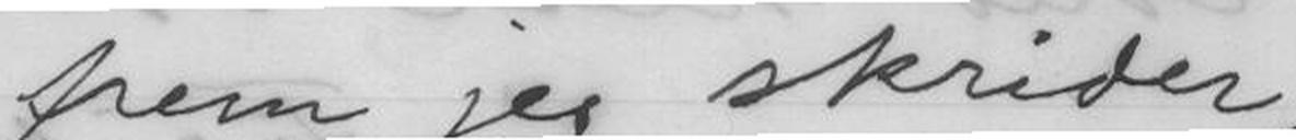frem jeg skrider.
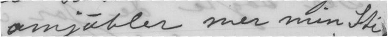omjubler mer min Sti;
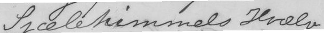Sjælehimmels Hvælv
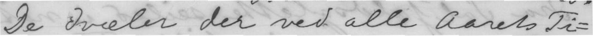De dvæler der ved alle Aarets Ti-
